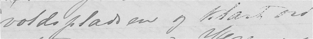voldspladsen og klart ori-
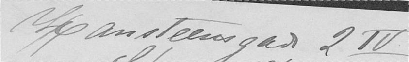Hansteens gade 2 IV
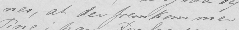nes, at der fremkommer
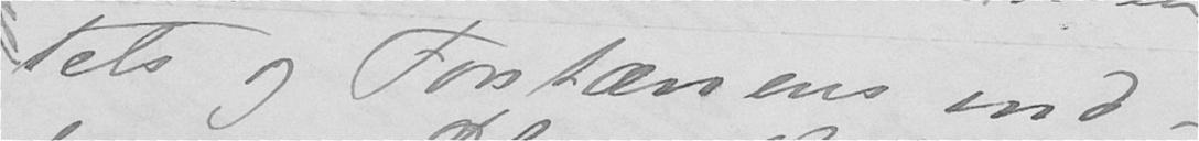tets og Fontænens ind-
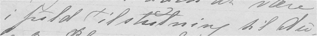i fuld Tilslutning til Au-
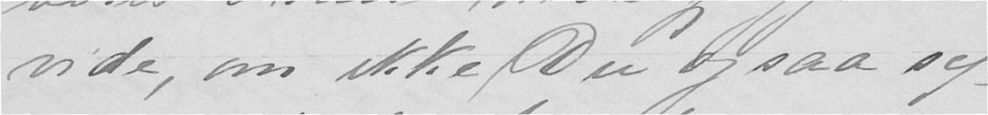vide, om ikke Du ogsaa sy-
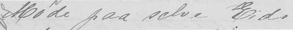Møde paa selve Eids-
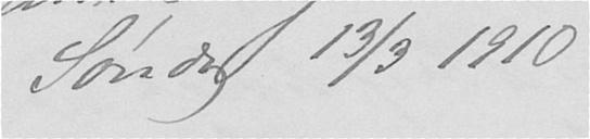Søndag 13/3 1910
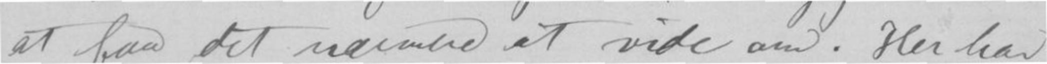at faa det nærmere at vide om. Her har
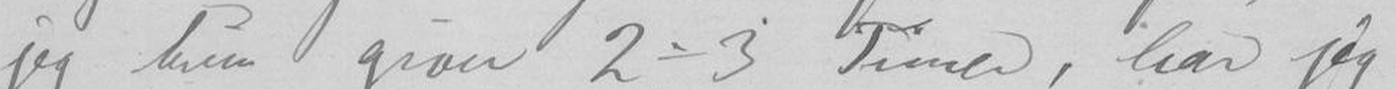jeg kun giver 2-3 Timer, har jeg
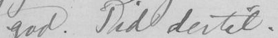god Tid dertil.
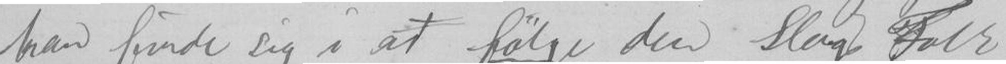kan finde sig i at følge den Slags Folk,
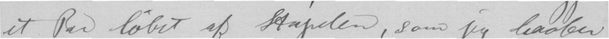et Par løbet af Stapelen, som jeg haaber
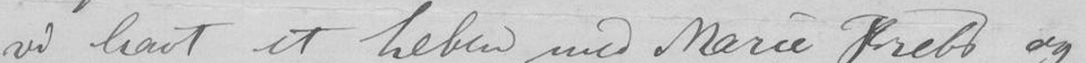vi havt et Leben med Marie Krebs og
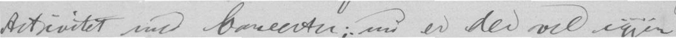Aktivitet med Concerten; nu er der vel igjen
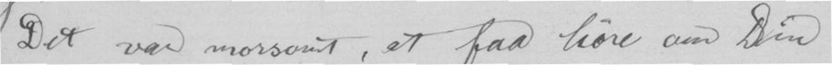Det var morsomt, at faa høre om Din
